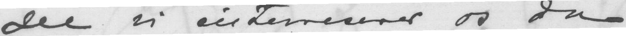see vi interreserer os da
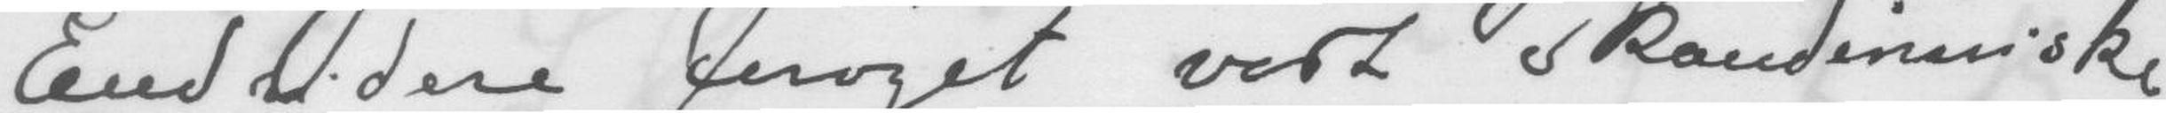Endvidere forøget vort skandinaviske
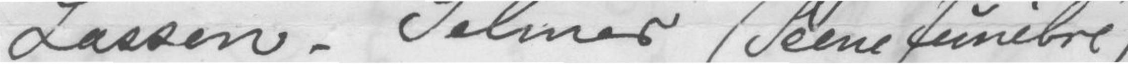Lassen - Selmer (Scene funibré)
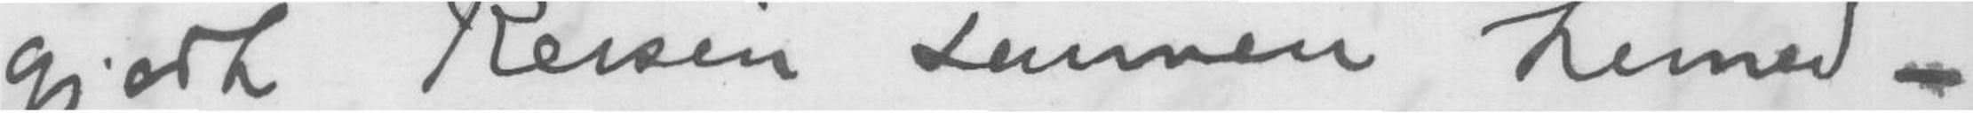gjort Reisen sammen hemad -
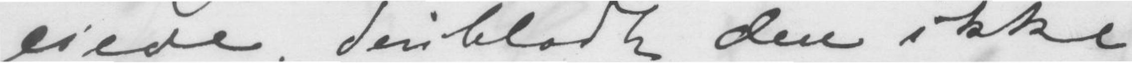eiede, deriblandt den ikke
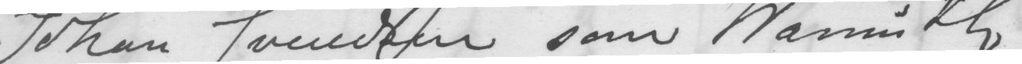Johan Svendsen som Warmuth
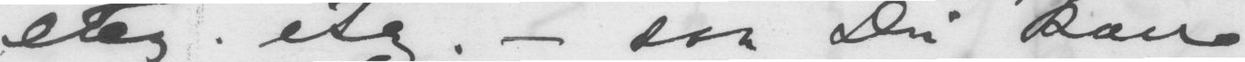etz. etz. - saa Du kan
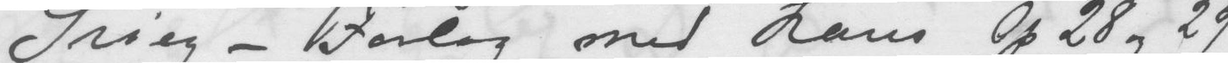Grieg-Forlag med hans Op 28 og 29
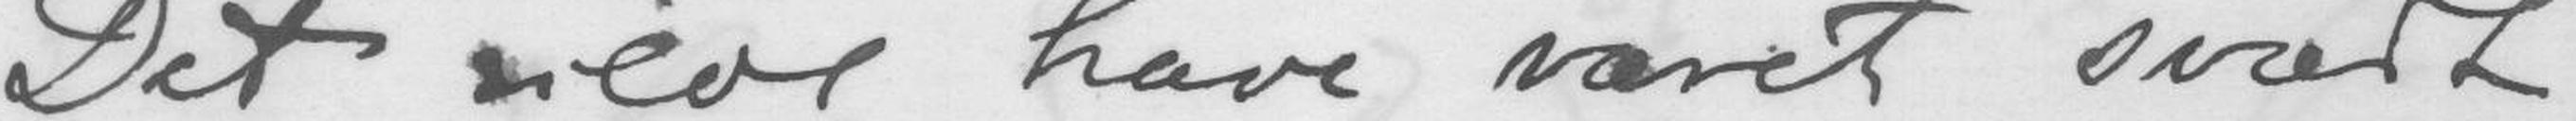Det vilde have været svært
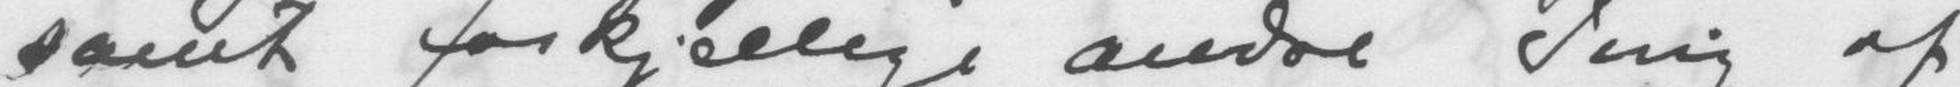samt forskjellige andre Ting af
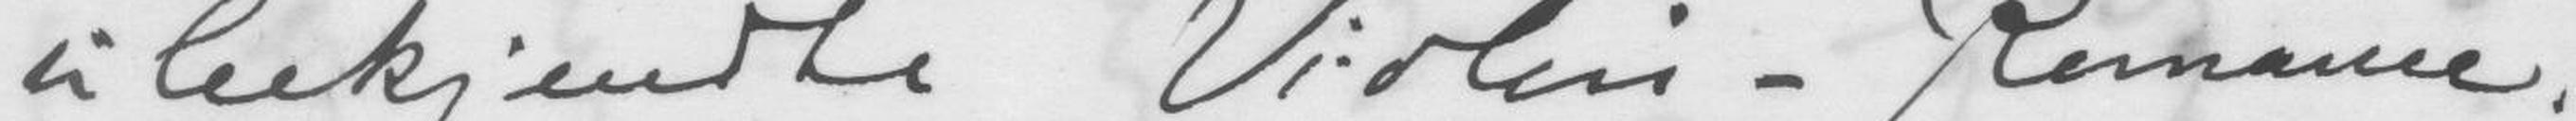ubekjendte Violin-Romance.
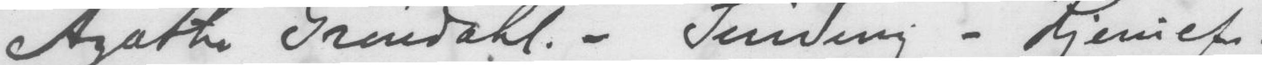Agathe Grøndahl. - Sinding - Kjerulf -
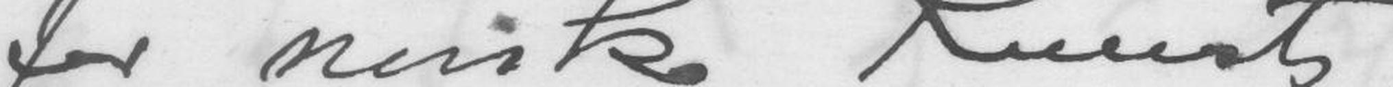for norsk Kunst.
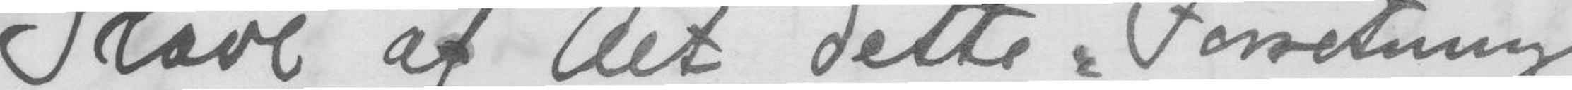Slave af Alt dette Forretnings
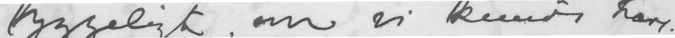hyggeligt, om vi kunde have
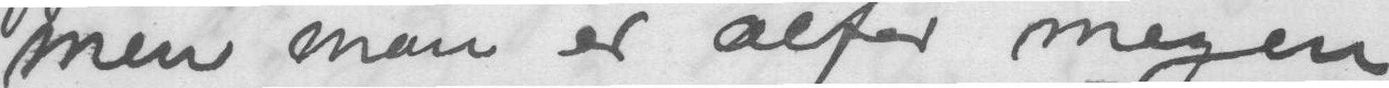men man er alfor megen
